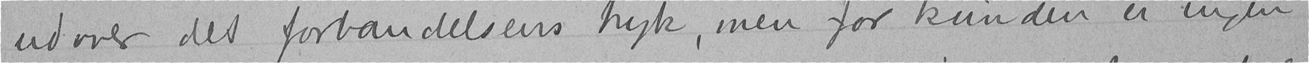udover det forbandelsens tryk, men for kvinden er ingen
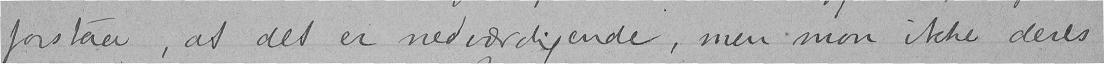forstaa, at det er nedværdigende, men mon ikke deres
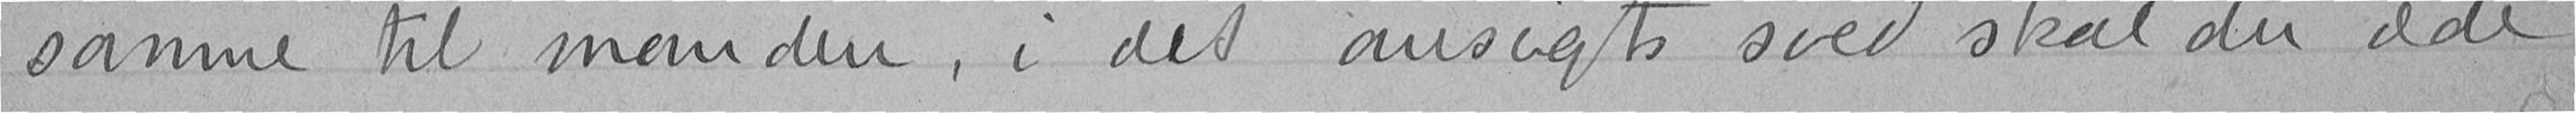samme til manden, i det ansigts sved skal du æde
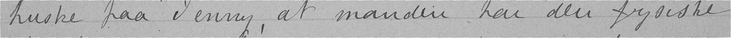huske paa Jenny, at manden har den fysiske
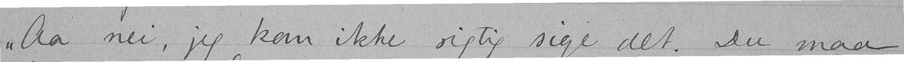"Aa nei, jeg kan ikke rigtig sige det. Du maa
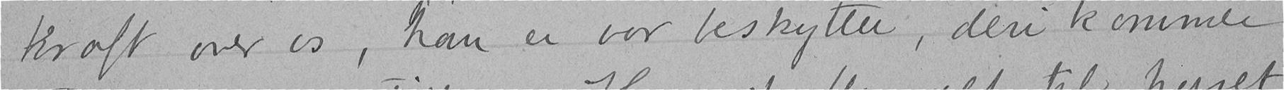kraft over os, han er vor beskytter, deri kommer
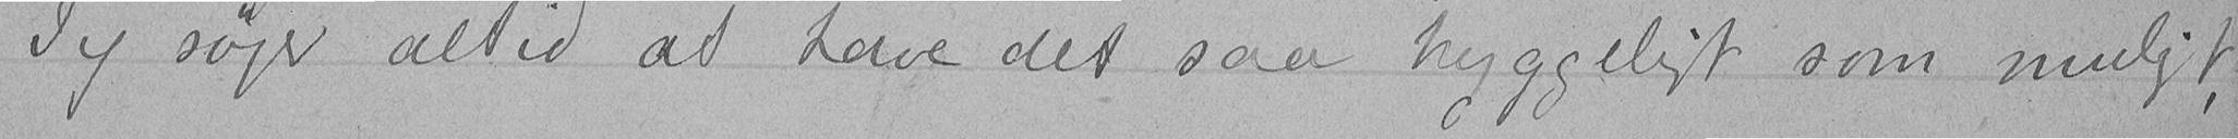Jeg søger altid at have det saa hyggeligt som muligt,
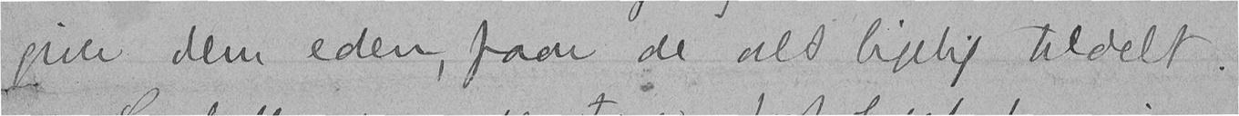giver dem eden, faar de alt ligelig tildelt.
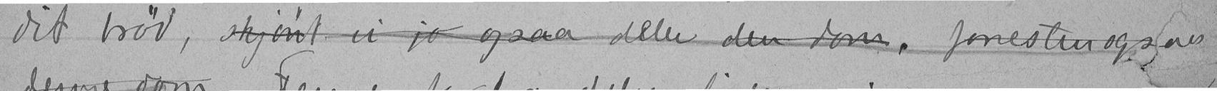dit brød, skjønt vi jo ogsaa deler den dom, forresten ogsaa
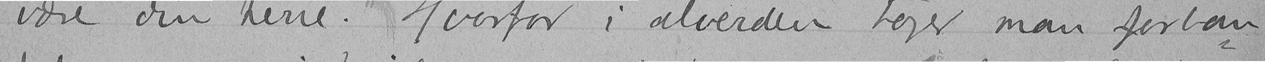være din herre. Hvorfor i alverden tager man forban-
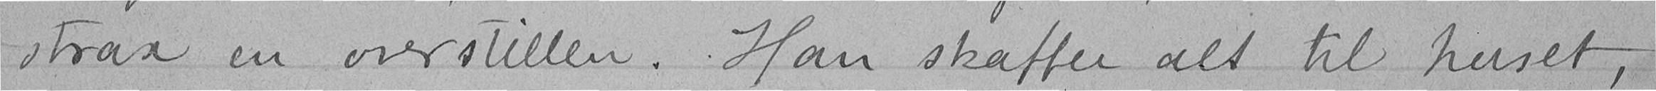strax en overstillen. Han skaffer alt til huset,
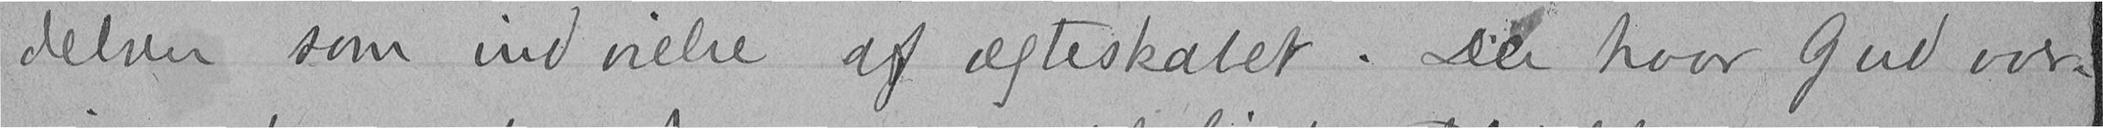delsen som indvielse af ægteskabet. Der hvor Gud over-
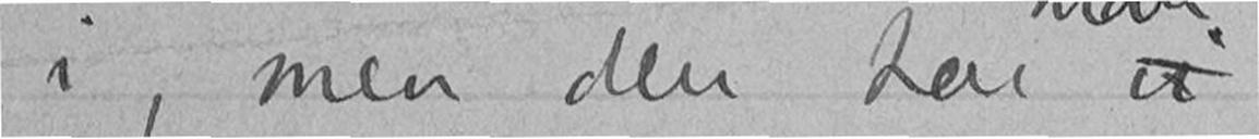i, men den har vi
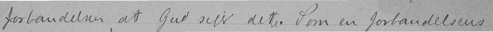forbandelsen at Gud siger dette. Som en forbandelsens
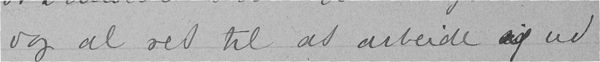dog al ret til at arbeide sig ud
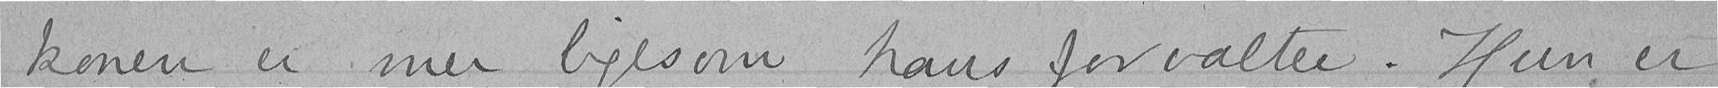konen er mer ligesom hans forvalter. Hun er
